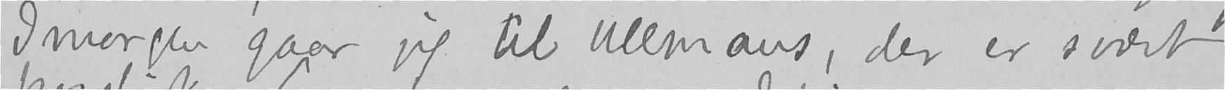Imorgen gaar jeg til Ullmans, der er svært
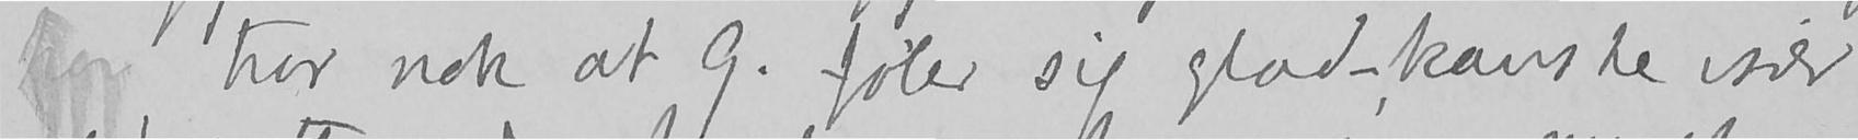har tror nok at G. føler sig glad - kanske især
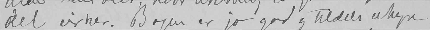del virker. Bogen er jo god og tildels uhyre
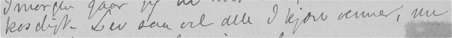koseligt. Lev saa vel alle I kjære venner, nu
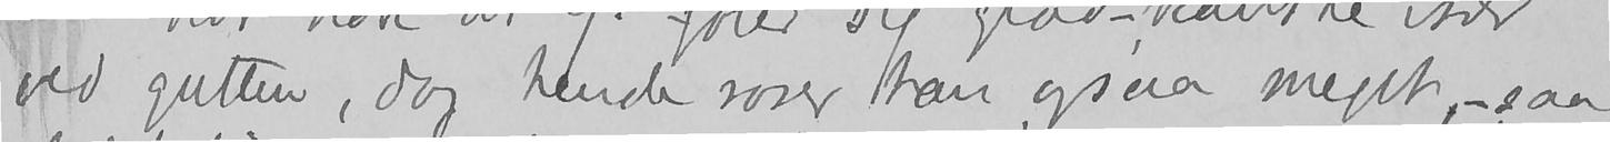ved gutten, dog hende roser han ogsaa meget, - saa
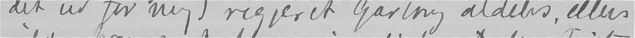det ud for mig) regjeret Garborg aldeles, ellers
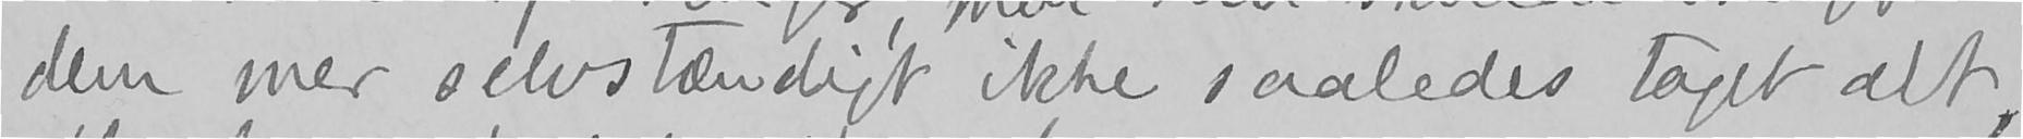dem mer selvstændigt ikke saaledes taget alt,
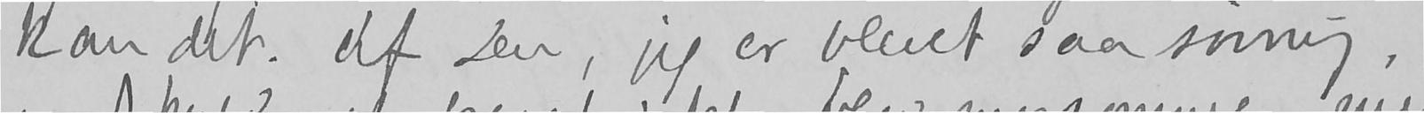kan det. Uf Du, jeg er blevet saa søvnig,
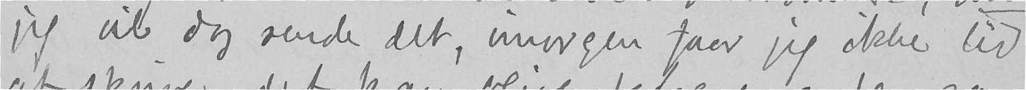jeg vil dog sende det, imorgen faar jeg ikke tid
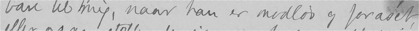bare til mig, naar han er modløs og foraset,
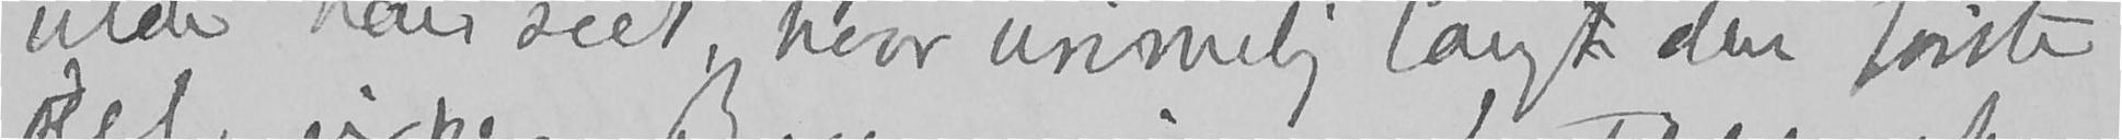vilde han seet, hvor urimelig langt den første
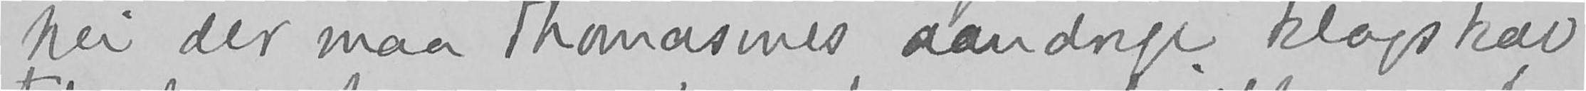Nei der maa Thomasines aandrige klogskab
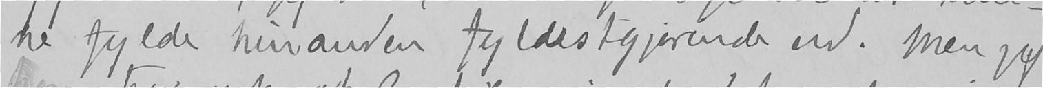ne fylde hinanden fyldestgjørende ud. Men jeg
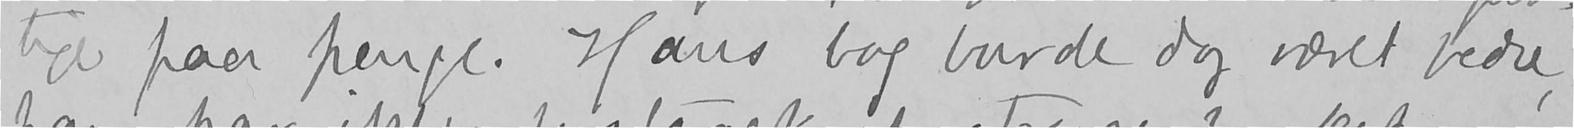tige paa penge. Hans bog burde dog været bedre,
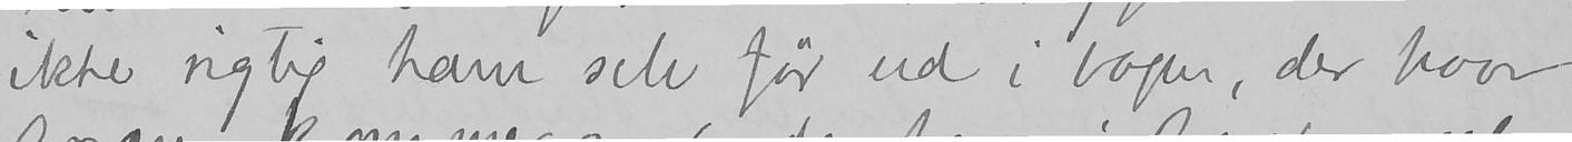ikke rigtig ham selv før ud i bogen, der hvor
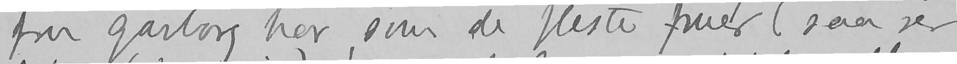fru Garborg har, som de fleste fruer (saa ser
